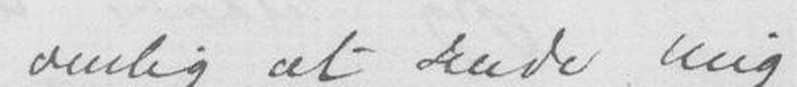venlig at sende mig
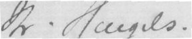Hr. Heugels.
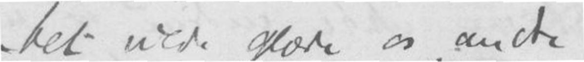Det vilde glæde os andre
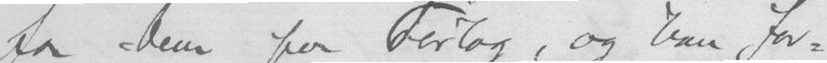faa Dem paa , og han for-
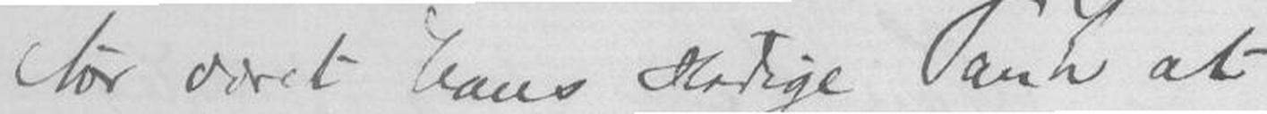aaret hans stadige Tanke at
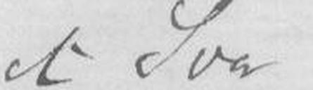et Svar
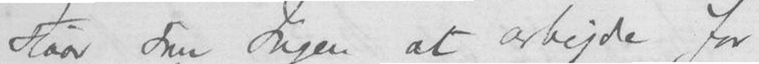staar Dem at arbeijde for
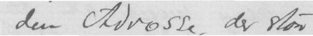den Adresse, der staar
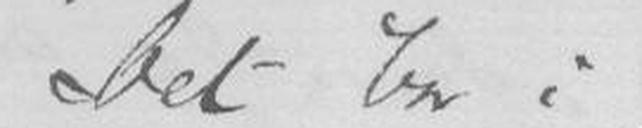Det har i
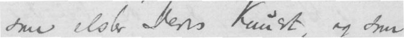som elsker Dens Kunst, og som
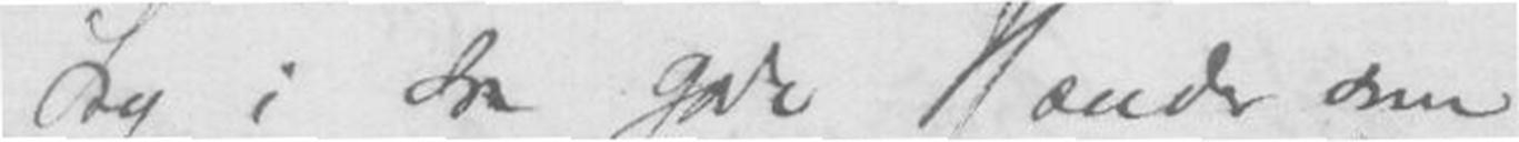sig i saa gode Hænder som
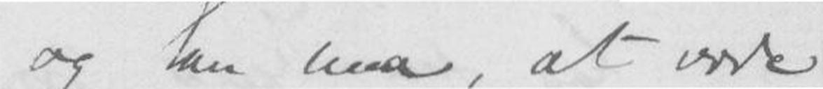og han maa, at være
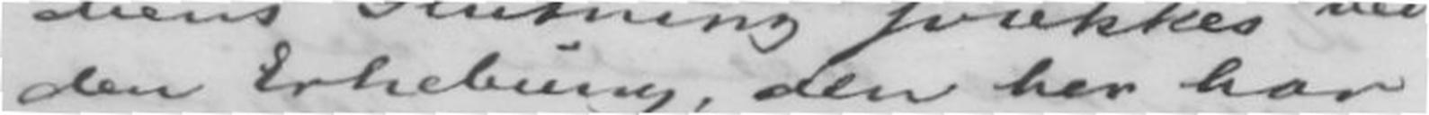den Erheburry, den her har
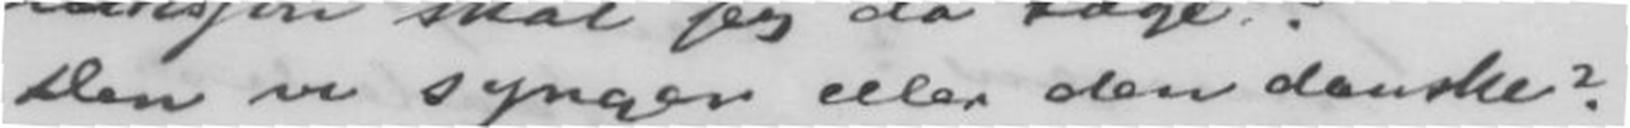Den vi synger eller den danske?
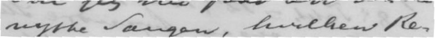nytte Sangen, hvilken Re-
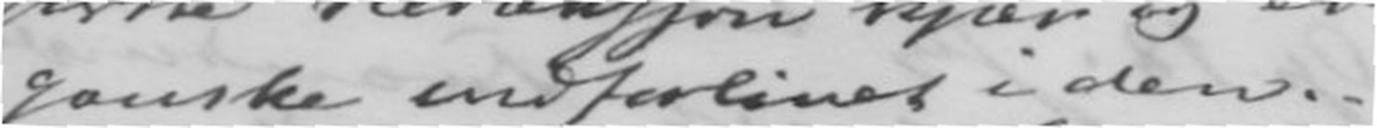ganske indforlivet i den. -
